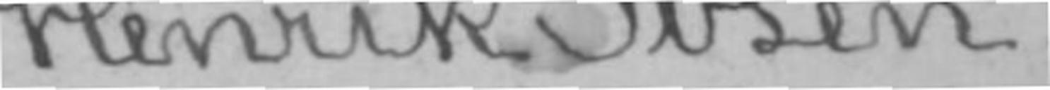Henrik Ibsen.
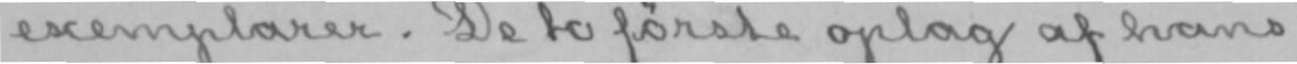exemplarer. De to første oplag af hans
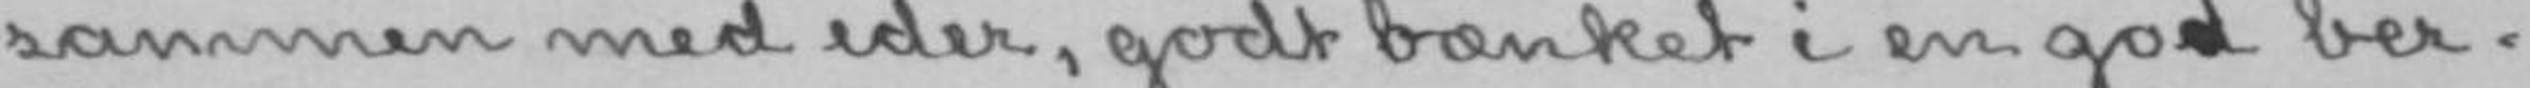sammen med eder, godt bænket i en god ber-
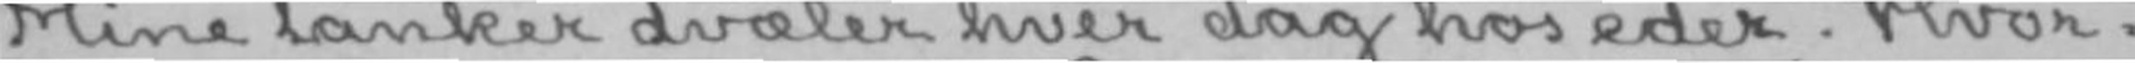Mine tanker dvæler hver dag hos eder. Hvor-
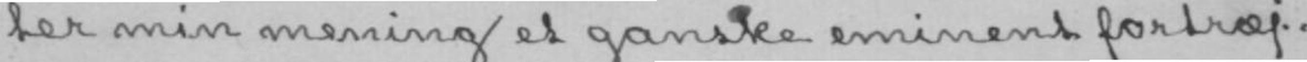ter min mening et ganske eminent fortræf-
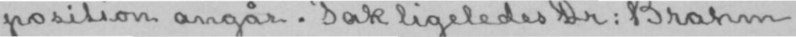position angår. Tak ligeledes Dr: Brahm
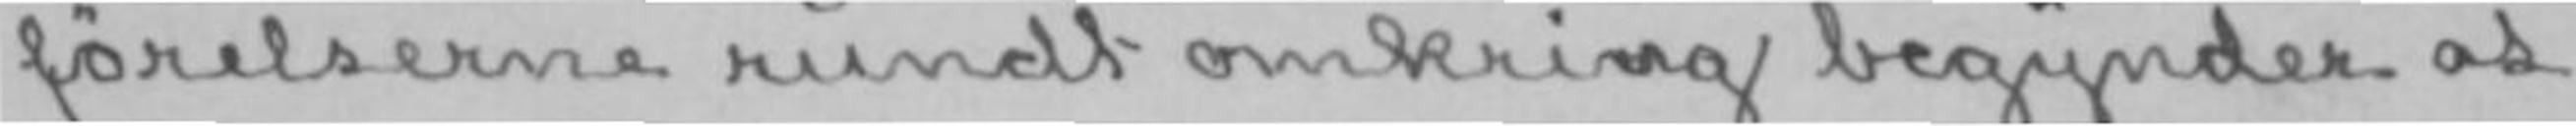førelserne rundt omkring begynder at
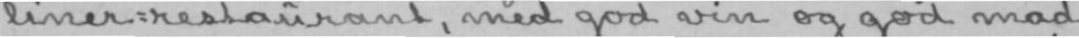liner-restaurant, med god vin og god mad
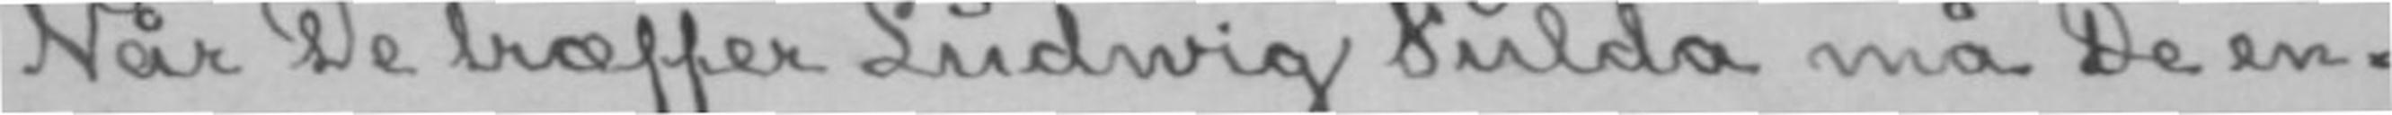Når De træffer Ludwig Fulda må De en-
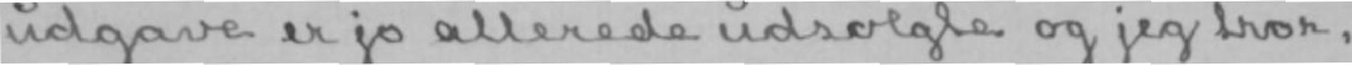udgave er jo allerede udsolgte og jeg tror,
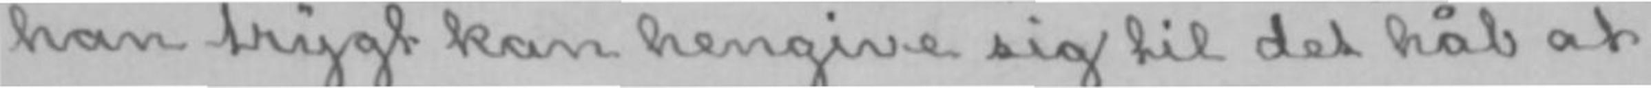han trygt kan hengive sig til det håb at
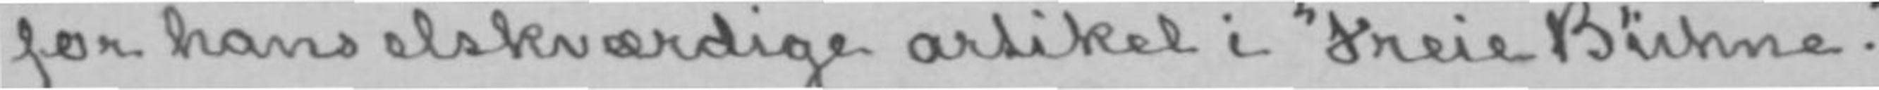for hans elskværdige artikel i «Freie Bühne».
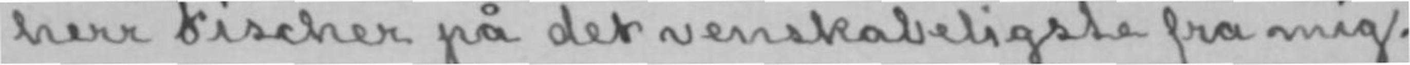herr Fischer på det venskabeligste fra mig.
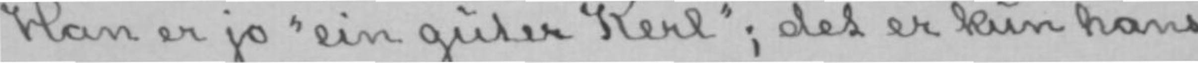Han er jo «ein guter Kerl»; det er kun hans
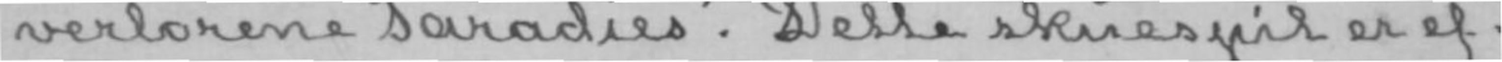verlorene Paradies». Dette skuespil er ef-
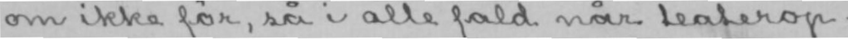om ikke før, så i alle fald når teaterop-
